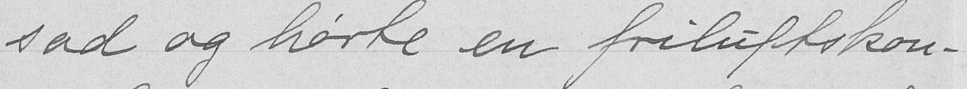sad og hørte en friluftskon-
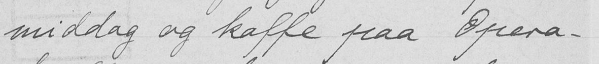middag og kaffe paa Opera-
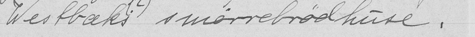Westbæks(?) smørrebrodhuse.
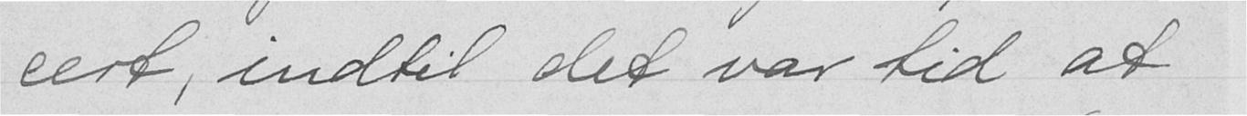cert, indtil det var tid at
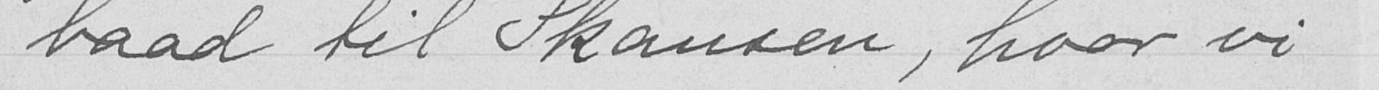baad til Skansen, hvor vi
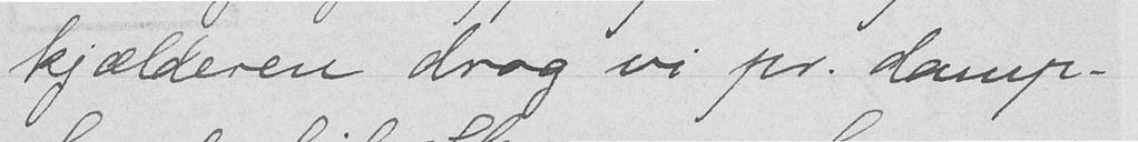kjælderen drog vi pr. damp-
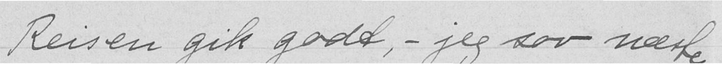Reisen gik godt, - jeg sov næsten
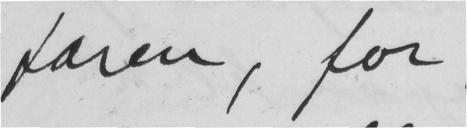faren, for
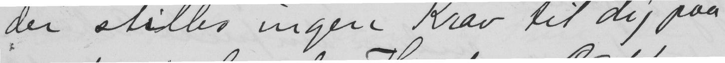der stilles ingen Krav til dig paa
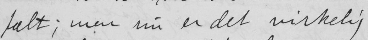fælt; men nu er det virkelig
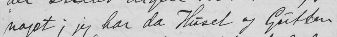noget; jeg har da Huset og Gutten
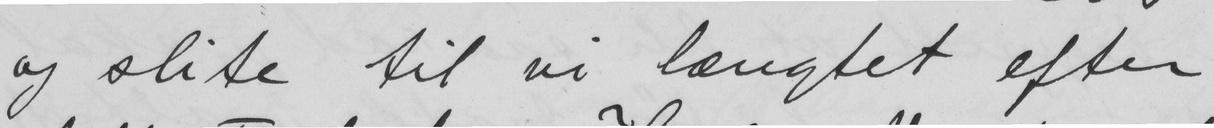og slite til vi længtet efter
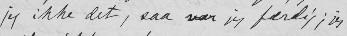jeg ikke det, saa var jeg færdig; jeg
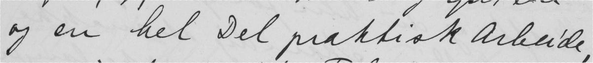og en hel Del praktisk arbeide
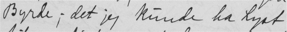Byrde; det jeg Kunde ha Lyst
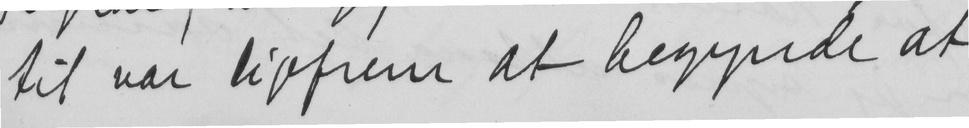til var ligfrem at begynde at
